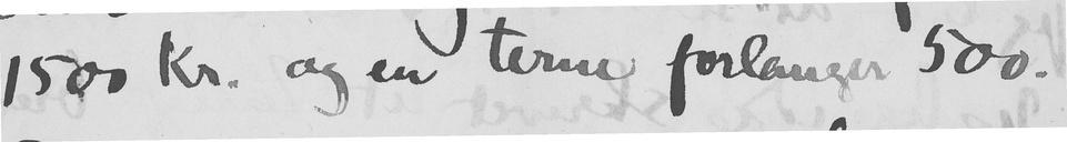1500 kr. og en terne forlanger 500.
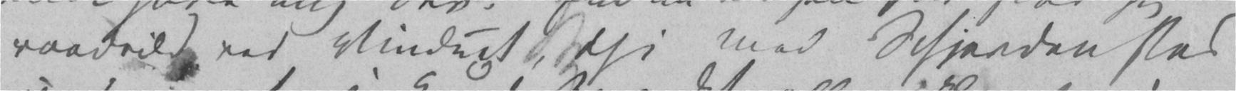raadvild ved Vinduet, thi med Schjander stod
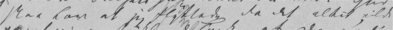skee Lov at jeg flyttede da det altid vilde
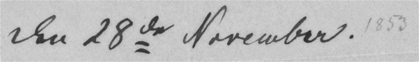Den 28de November.
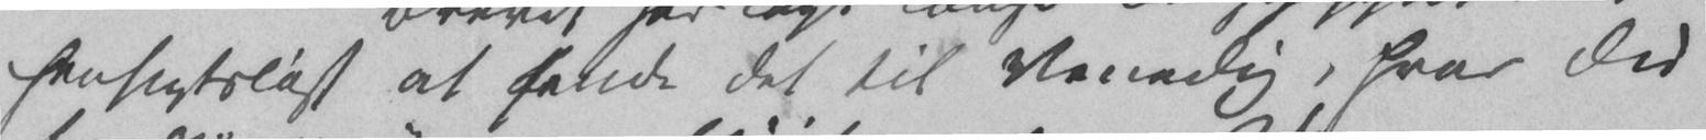hensigtsløst at sende det til Venedig, hvor Du
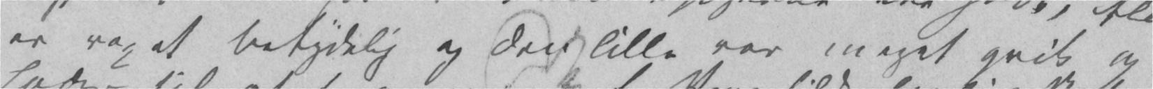er voxet betydelig og den lille var meget qvik og
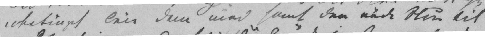ubetinget leie dem med samt den røde Stue til
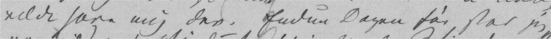vilde have mig der. Endnu Dagen før stod jeg
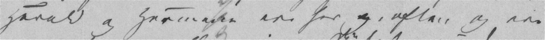Harald og Hermana ere her og i aften og ere
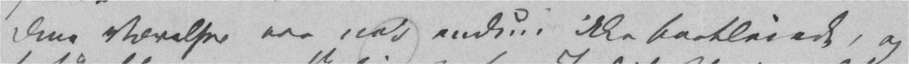Dine Værelser ere nok endnu ikke bortleiede, og
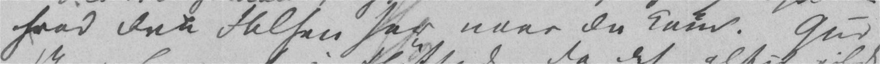hvad Fru Falsen har naar Du kom. Gud
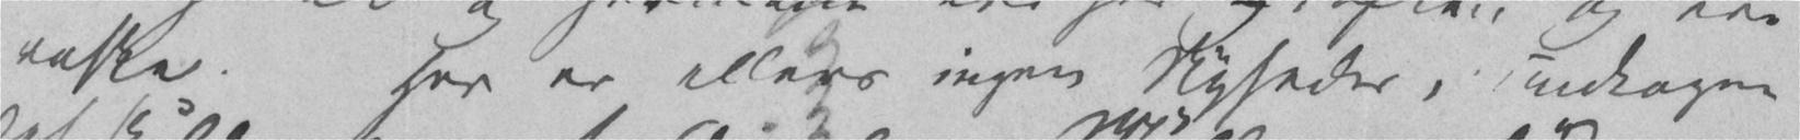raske. Her er ellers ingen Nyheder, undtagen
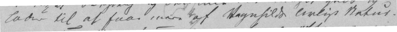lader til at faae mere af Ragnhilds livlige Natur.
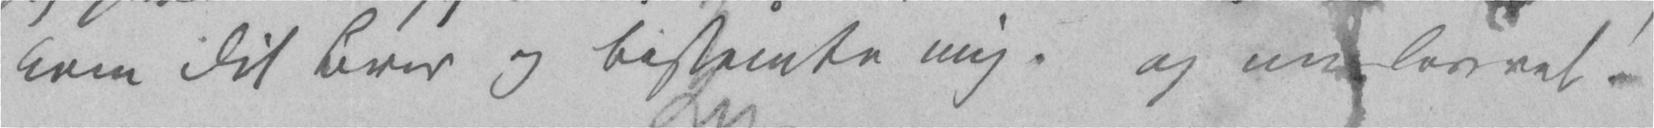kom Dit Brev og bestemte mig. og nu lev vel!
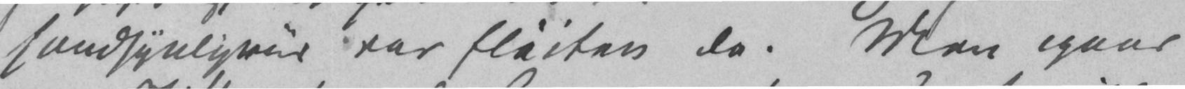sandsynligviis var fløiten da. Men igaar
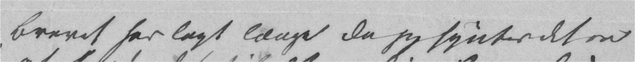Brevet har lagt længe da jeg syntes det er
